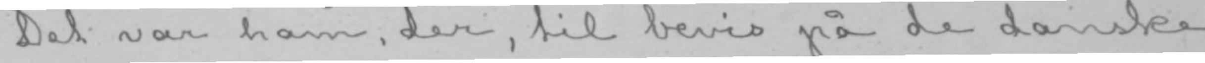Det var ham, der, til bevis på de danske
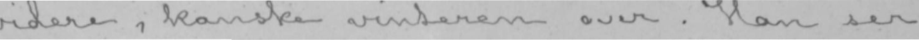videre, kanske vinteren over. Han ser
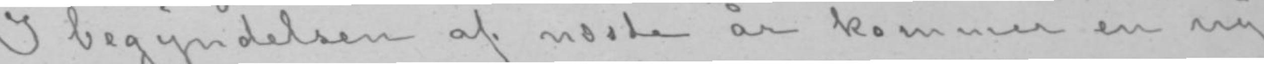I begyndelsen af næste år kommer en ny
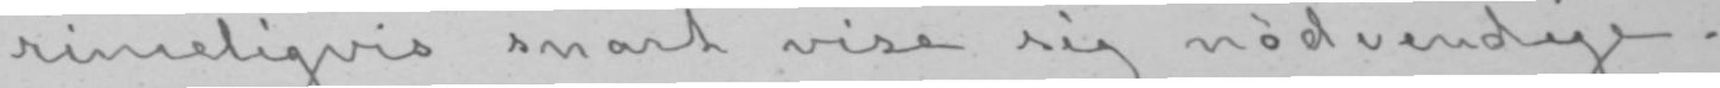rimeligvis snart vise sig nødvendige.
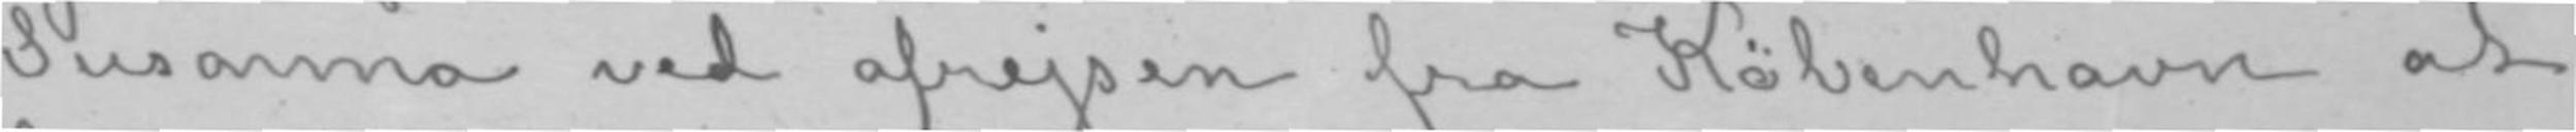Susanna ved afrejsen fra København at
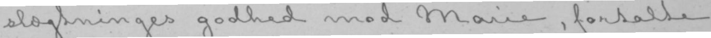slægtninges godhed mod Marie, fortalte
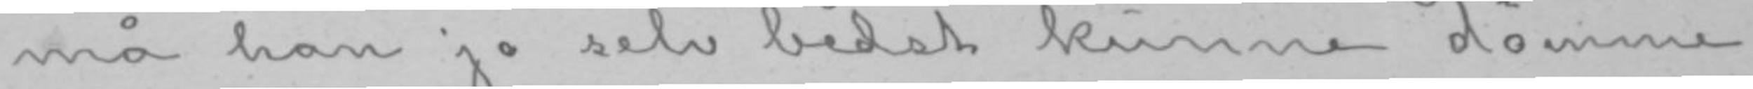må han jo selv bedst kunne dømme.
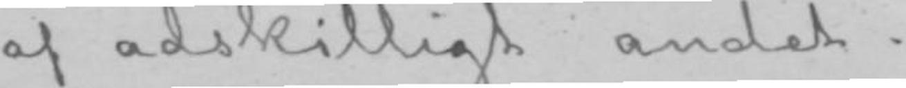af adskilligt andet.
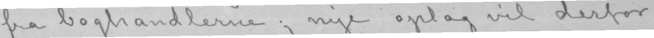fra boghandlerne; nye oplag vil derfor
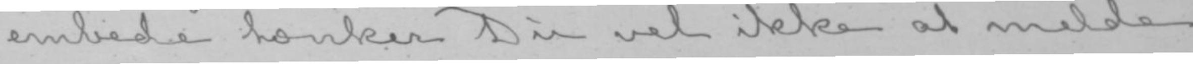embede tænker Du vel ikke at melde
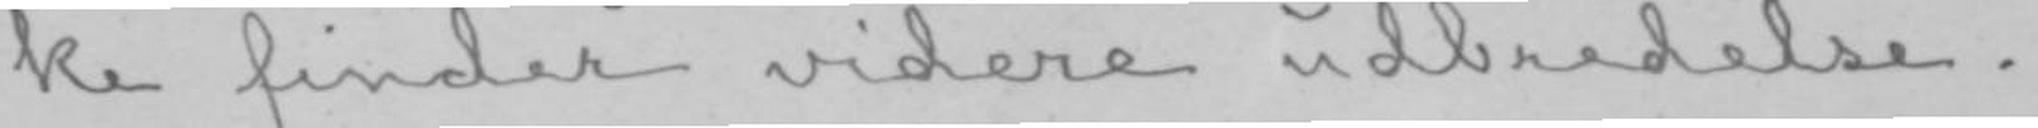ke finder videre udbredelse.
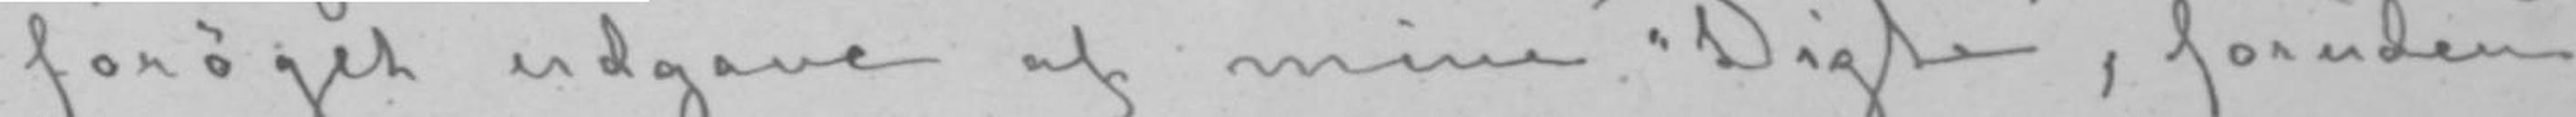forøget udgave af mine «Digte», foruden
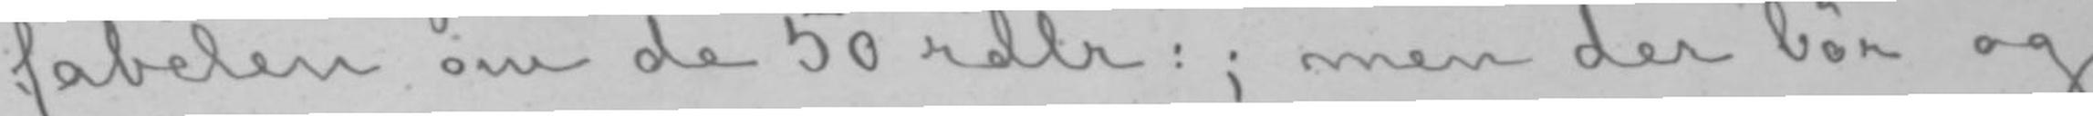fabelen om de 50 rdlr:; men der bør og
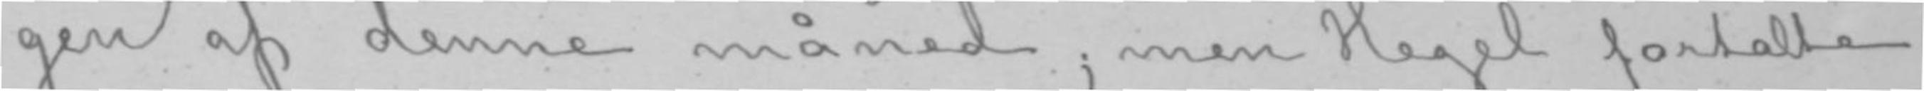gen af denne måned; men Hegel fortalte
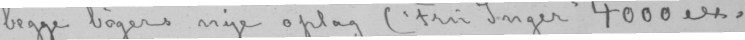begge bøgers nye oplag («Fru Inger» 4000 ex-
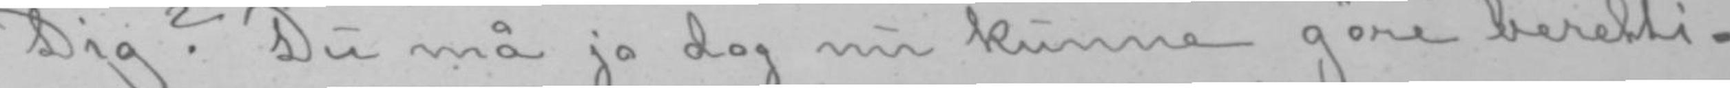Dig? Du må jo dog nu kunne gøre beretti-
